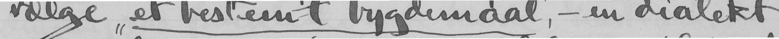vælge "et bestemt bygdemaal", - en dialekt
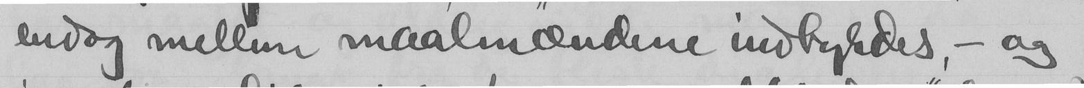endog mellem maalmændene indbyrdes - og
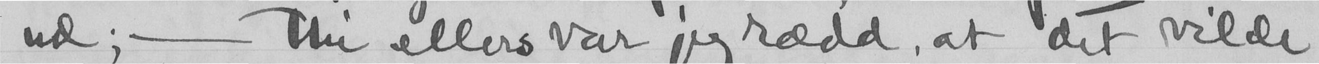ud; - thi ellers var jeg rædd, at det vilde
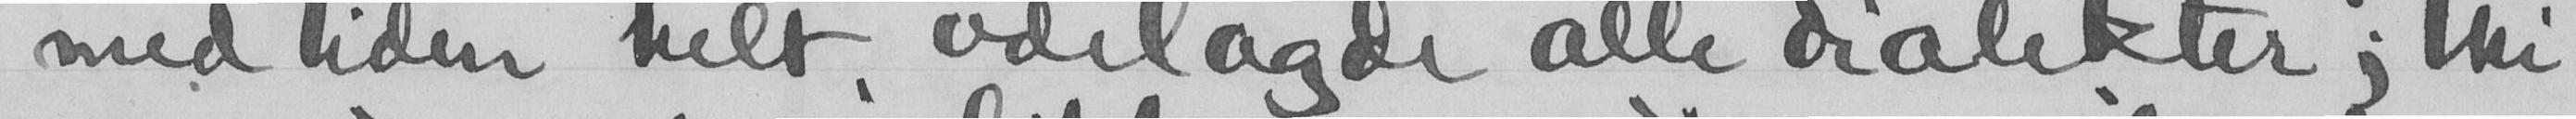med tiden helt ødelagde alle dialekter; thi
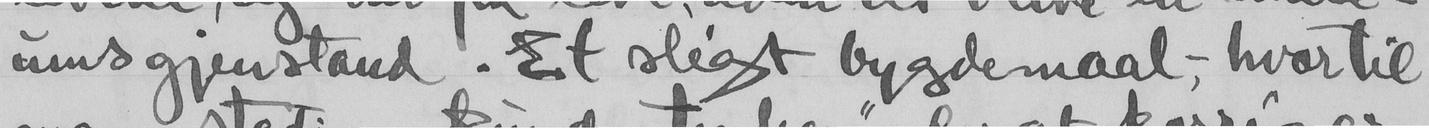umsgjenstand. Et sligt bygdemaal, - hvortil
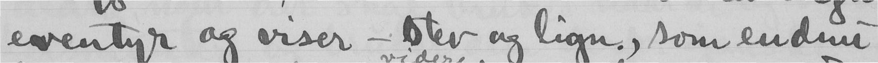eventyr og viser - stev og lign., som endnu
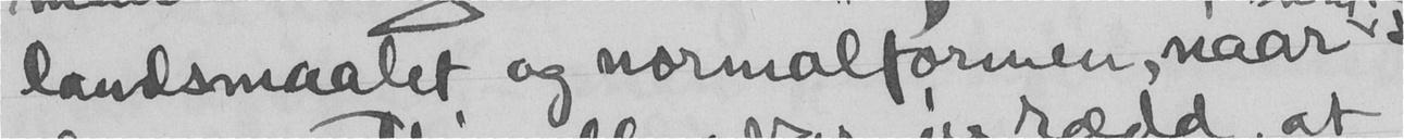landsmaalet og normalformen, naar
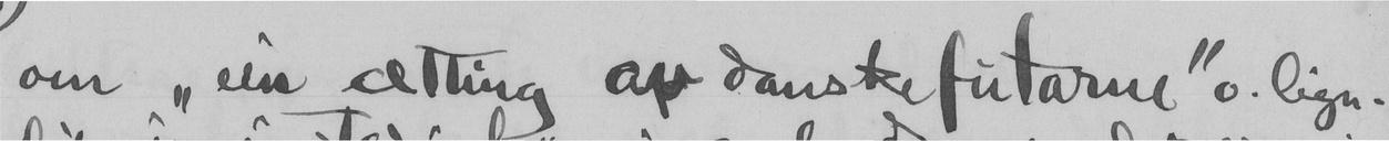om "ein ætting af danskefutarne" o. lign.
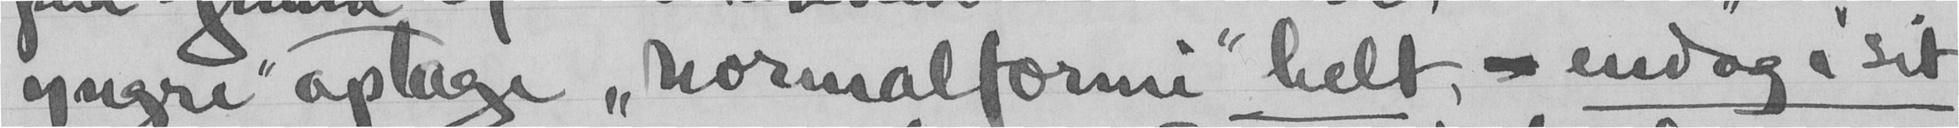yngre" optage "normalformi" helt, - endog i sit
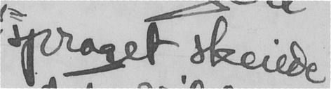sproget skeiede
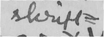skrift-
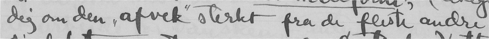dig om den "afvek" sterkt fra de fleste andre
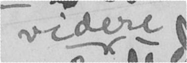videre
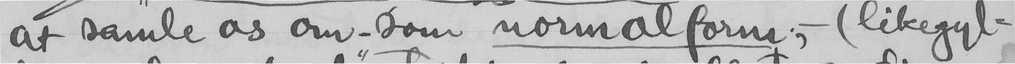at samle os om - som normalform; - (likegyl-
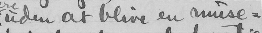uden at blive en muse-
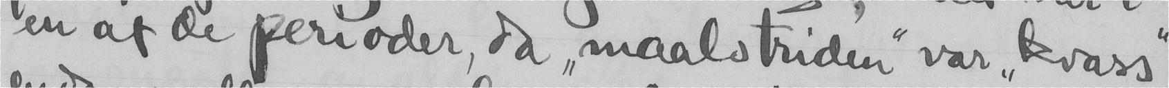en af de perioder, da "maalstriden" var "kvass"
